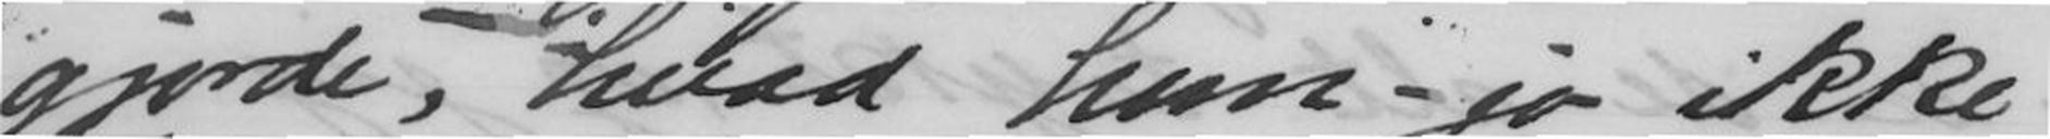gjorde, hvad hun jo ikke
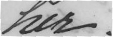her.
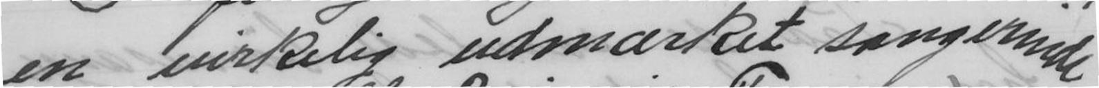en virkelig udmærket sangerinde
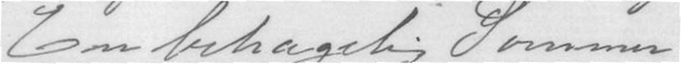En behagelig Sommer
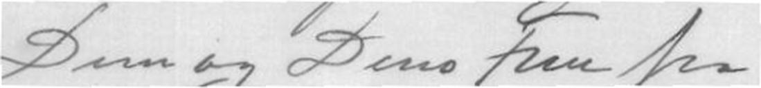Dem og Deres Frue fra
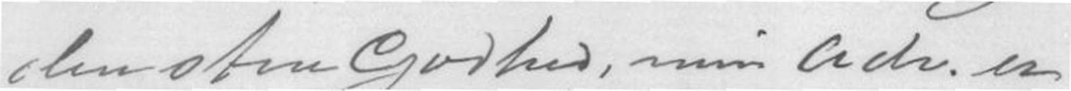den stor Godhed, min Adr. er
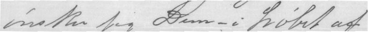ønsker jeg Dem - i Løbet af
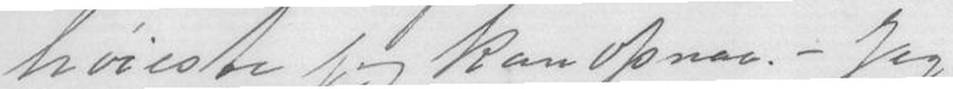høieste jeg kan opnaa. - Jeg
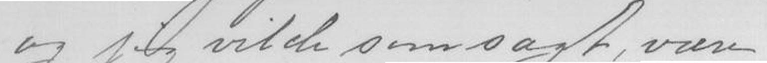og jeg vilde som sagt, være
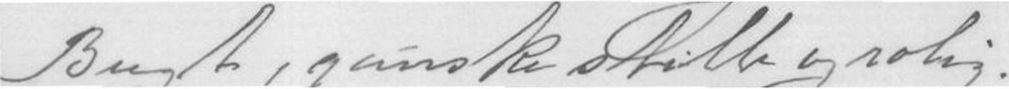Bugt, ganske stille og rolig.
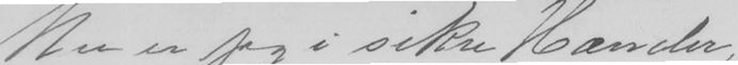Nu er jeg i sikre Hænder,
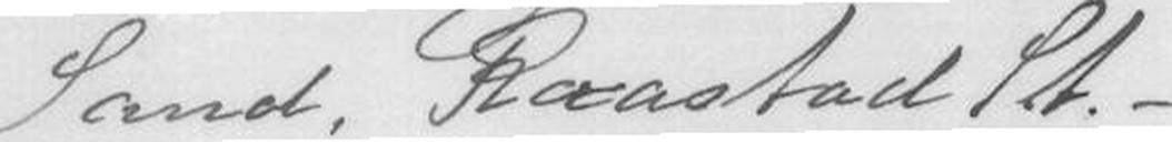Sand, Raastad St. -
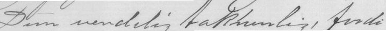Dem uendelig taknemlig, fordi
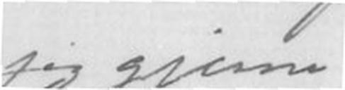jeg gjerne
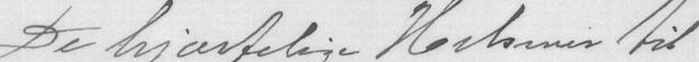De hjærtelige Hilsener til
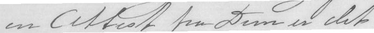en Attest fra Dem er det
